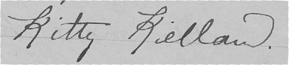Kitty Kielland.
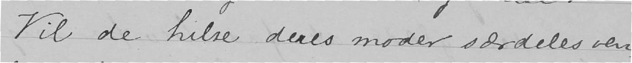Vil de hilse deres moder særdeles ven-
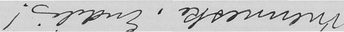menneske. Endelig!
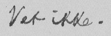Vet ikke.
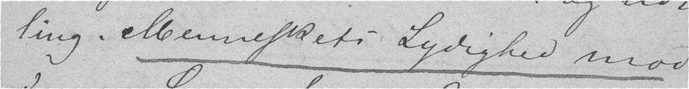ling. Menneskets Lydighed mod
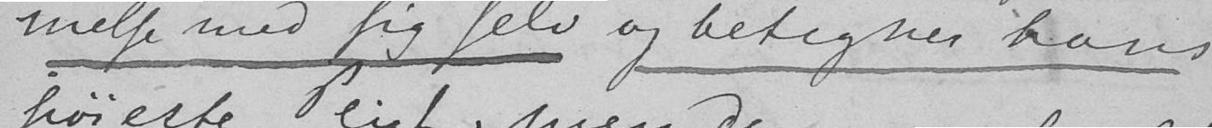melse med sig selv og betegner hans
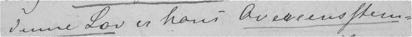denne Lov er hans Overeensstem-
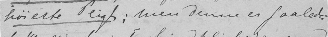høieste Pligt; men denne er saaledes
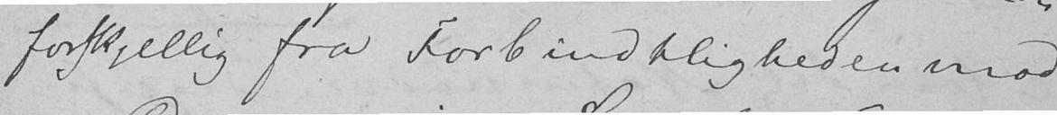forskjellig fra Forbindtligheden mod
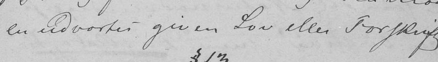en udvortes given Lov eller Forskrift.
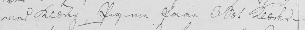med Klæder, Pigerne faar 3 Sæt Klæder -
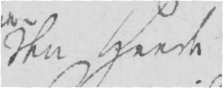den Heede
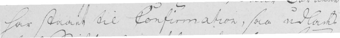har staaet til Confirmation, saa udlades
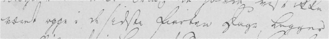været oppe i de sidste fiorten Dage, legger
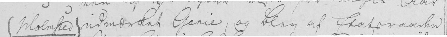(Malmsted) udmærket Genie, og blev af Etatsraaden
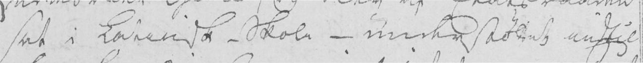sat i Latinsk-Skole - understøttet indtil
